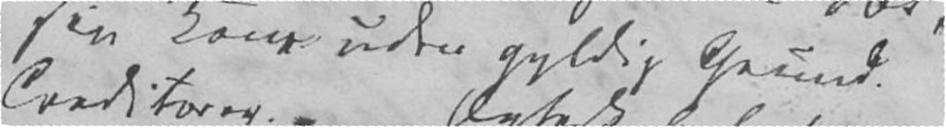sin Kone uden gyldig Grund.
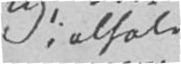ialfald
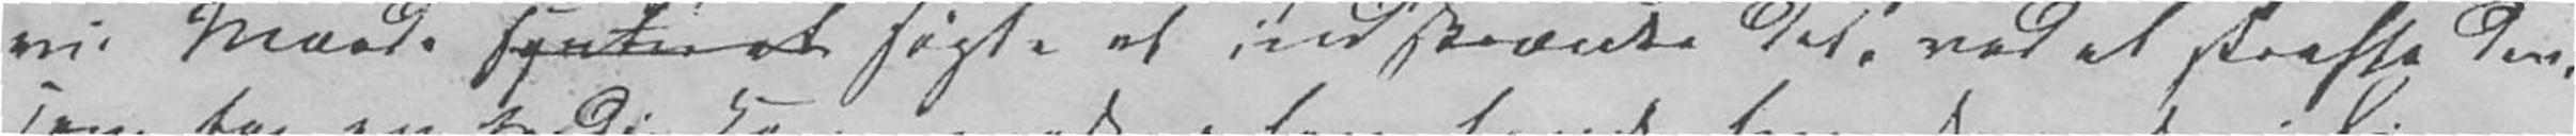vis Maade syntes at søgte at indskrænke det, ved at straffe den,
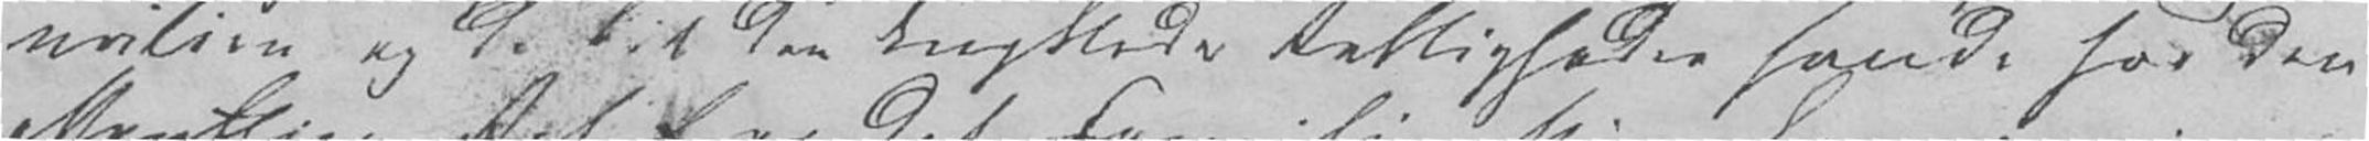milien og de til den knyttede Rettigheder havde for den
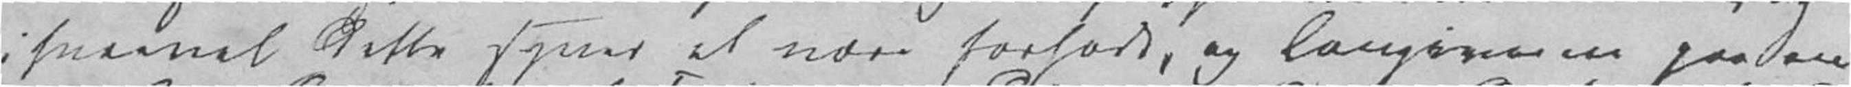ihvorvel dette synes at være forladt, og Lovgivningen paa en
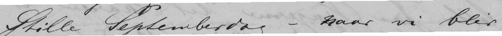stille Septemberdag - naar vi blir
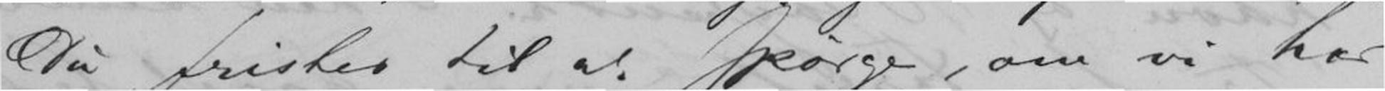Du fristes til at spørge, om vi har
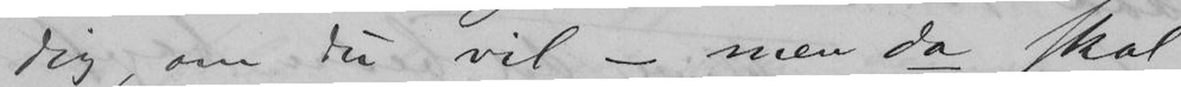dig, om Du vil - men da skal
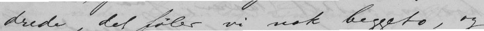drede, det føler vi nok beggeto, og
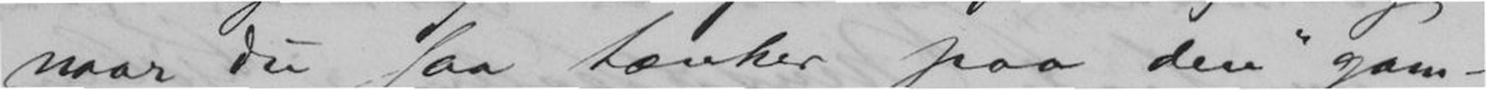naar Du saa tænker paa den "gam-
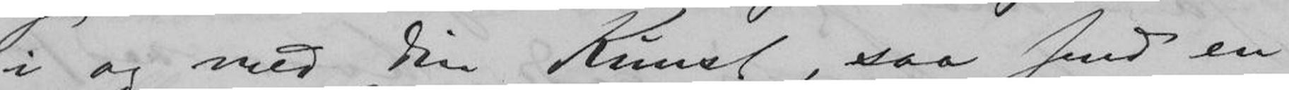i og med din Kunst, saa send en
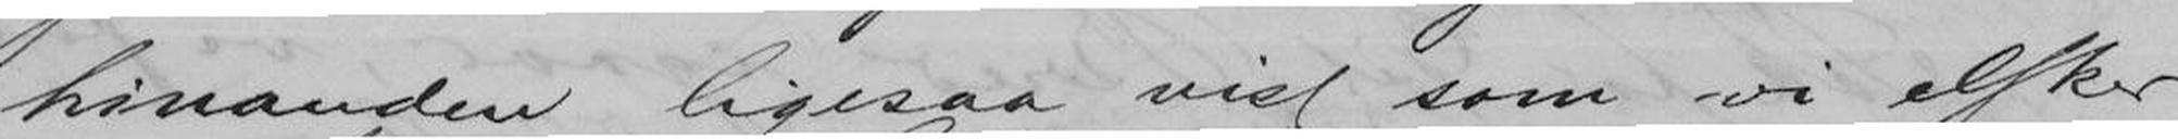hinanden ligesaa vist som vi elsker
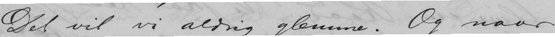Det vil jeg aldrig glemme. Og naar
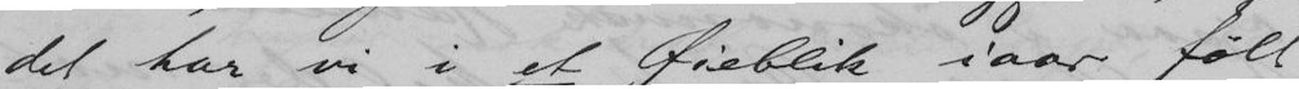det har vi i et Øieblik iaar følt
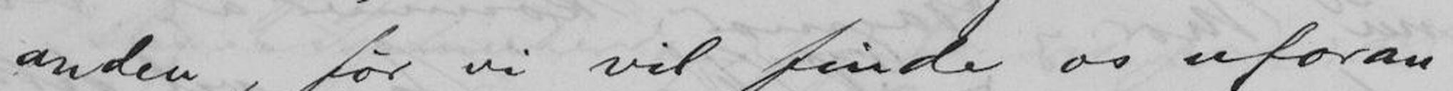anden, før vi vil finde os uforan-
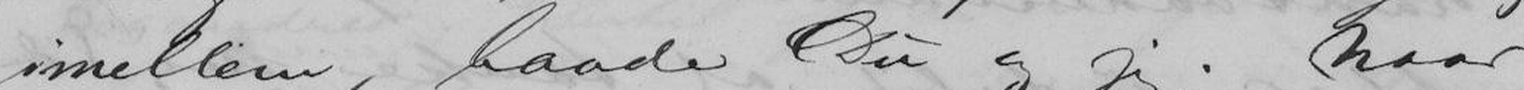imellem, baade Du og jeg. Naar
